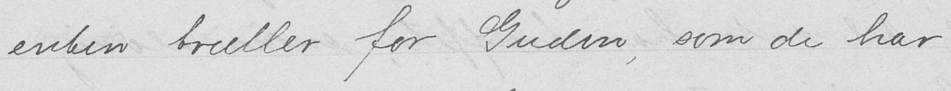enten træller for Guden, som de har
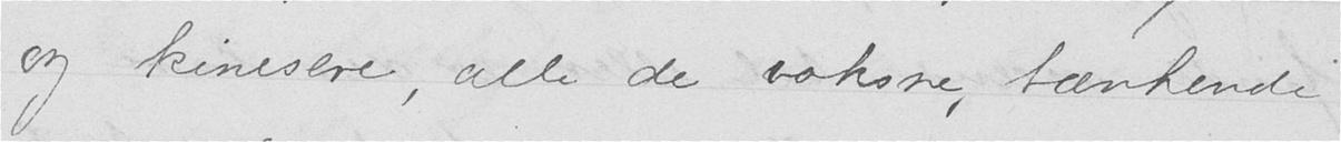og kinesere, alle de voksne, tænkende
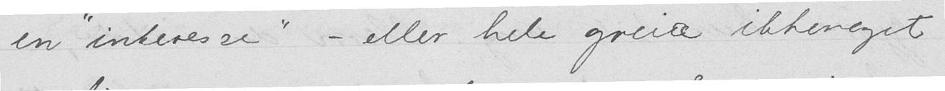en "interesse" - eller hele greia ikkenoget
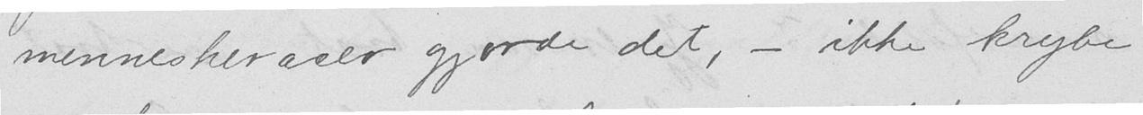menneskeracer gjorde det, - ikke krybe
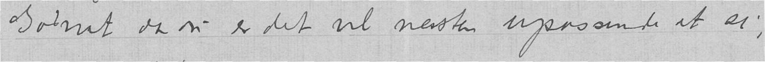Godnat da du er det vel nessten upassende at si,
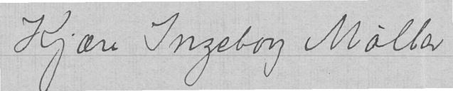Kjære Ingeborg Møller
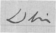Din
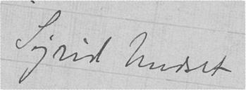Sigrid Undset
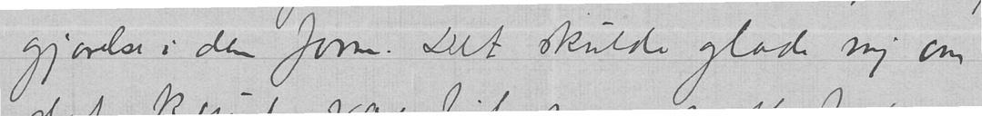gjørelse i den form. Det skulde glæde mig om
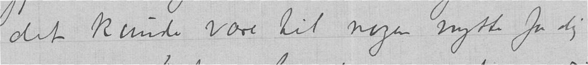det kunde være til nogen nytte for dig
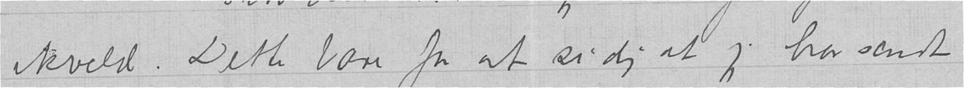ikveld. Dette bare for at si dig at jeg har sendt
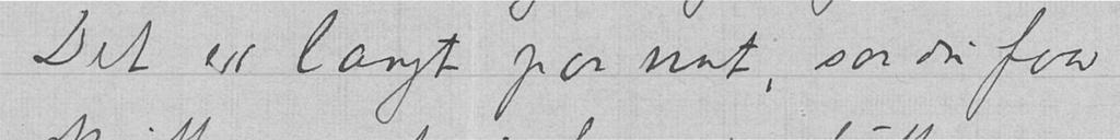Det er langt paa nat, saa du faar
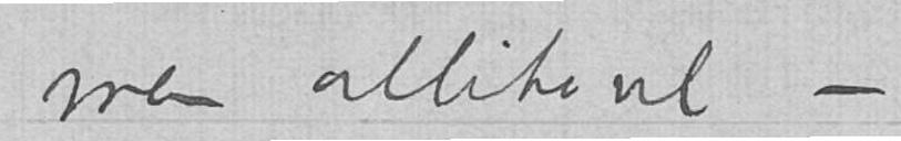men allikevel -
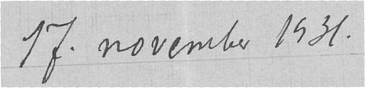17. november 1931.
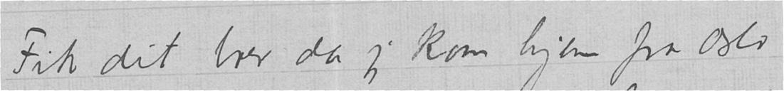Fik dit brev da jeg kom hjem fra Oslo
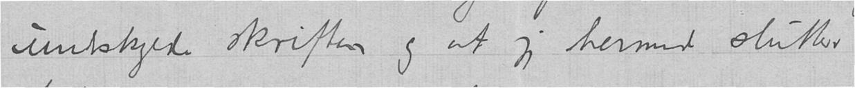undskylde skriften og at jeg hermed slutter
